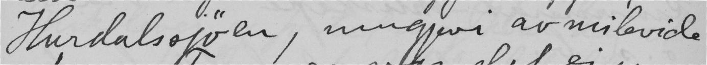Hurdalsjöen, umgjevi av milevide
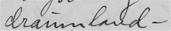draumland –
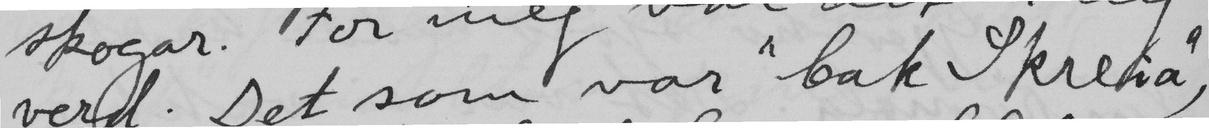verd. Det som var "bak Skreia",
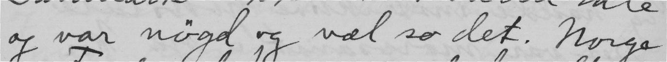og var nögd og væl so det. Norge
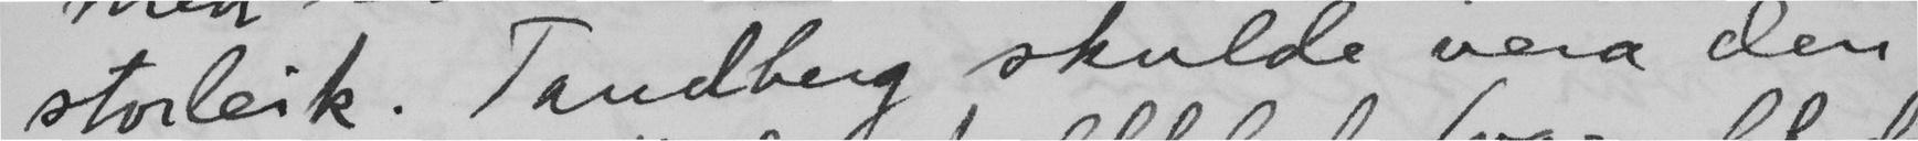storleik. Tandberg skulde vera den
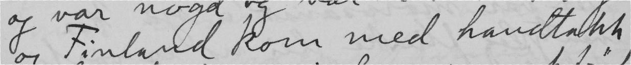og Finland kom med handtakk
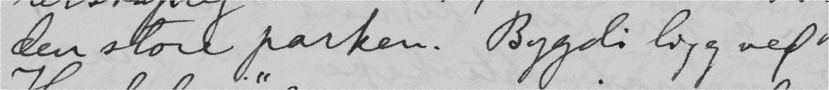den store parken. Bygdi ligg ved
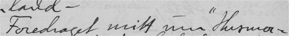Foredraget mitt um "Husmor-
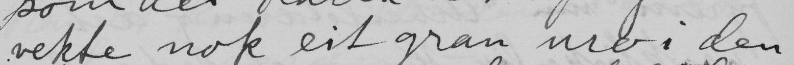vekte nok eit gran uro i den
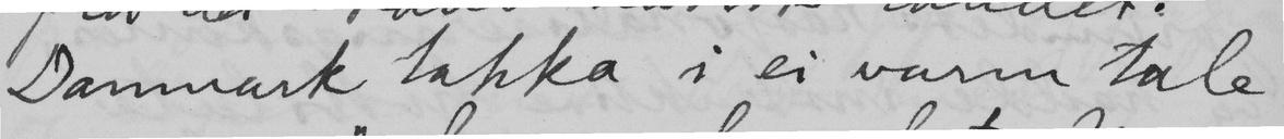Danmark takka i ei varm tale
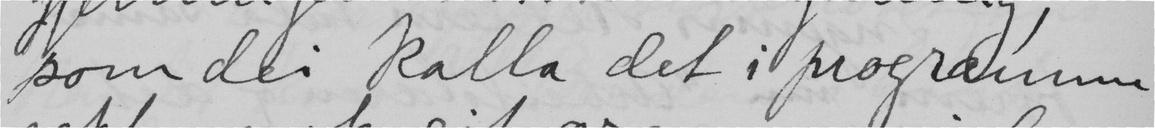som dei kalla det i pogramme
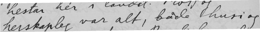herskapleg var alt, både husi og
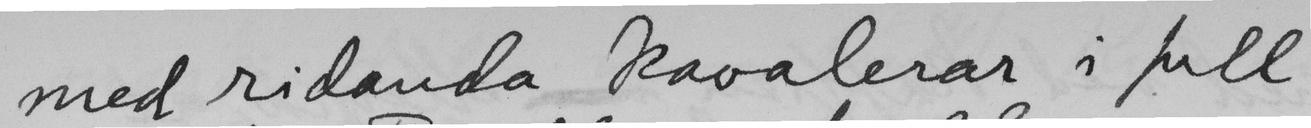med ridanda kavalerar i full
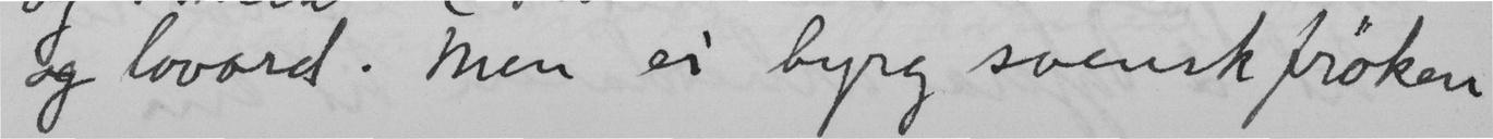og lovord. Men ei byrg svensk fröken
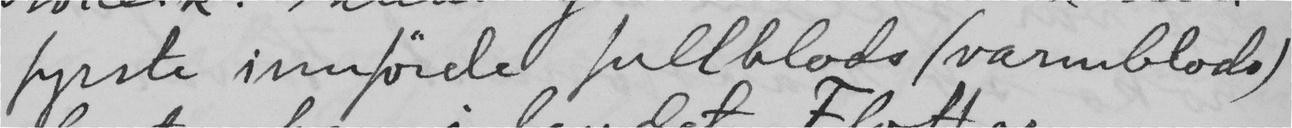fyrste innförde fullblods (varmblods)
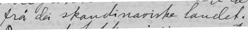frå dei skandinaviske landet.
